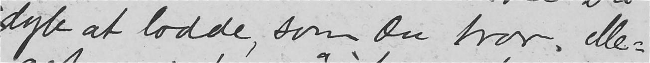dyb at lodde, som Du tror. Me-
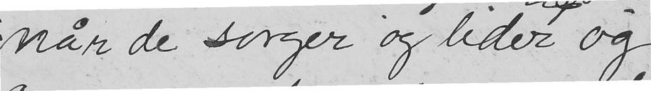når de sorger og leder og
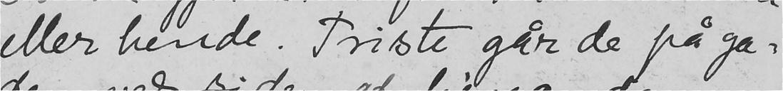eller hende. Triste går de på ga-
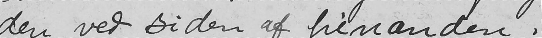den ved siden af hinanden.
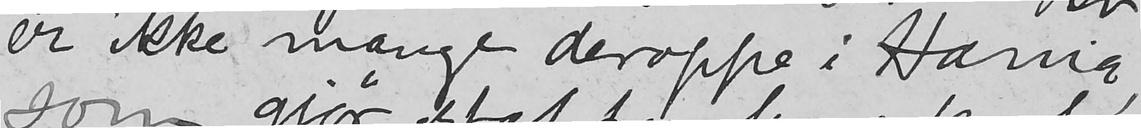er ikke mange deroppe i Xania,
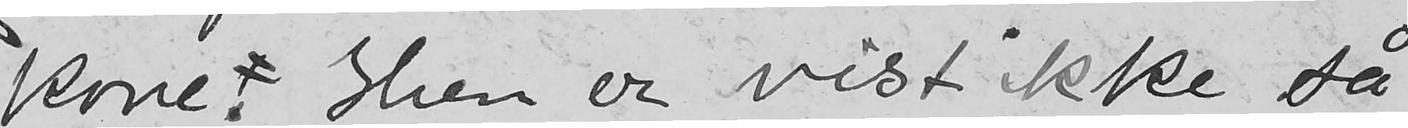kone. Hun er vist ikke så
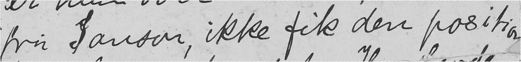fri Janson, ikke fik den position
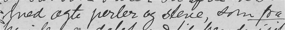med ægte perler og stene, som fra
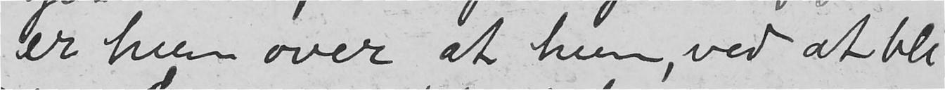er hun over at hun, ved at bli
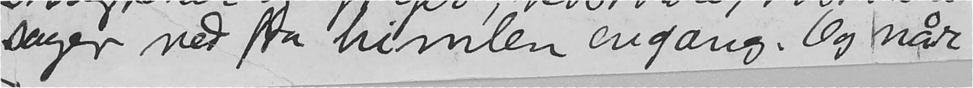sager ned fra himlen engang. Og når
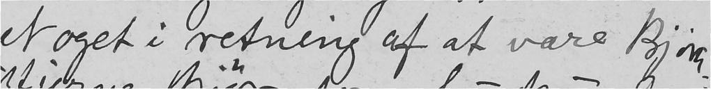Noget i retning af at være Bjørn-
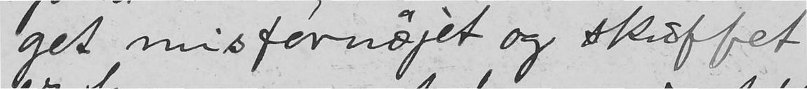get misfornøjet og skuffet
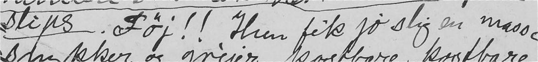slips. Føj!! Hun fik jo slig en masse
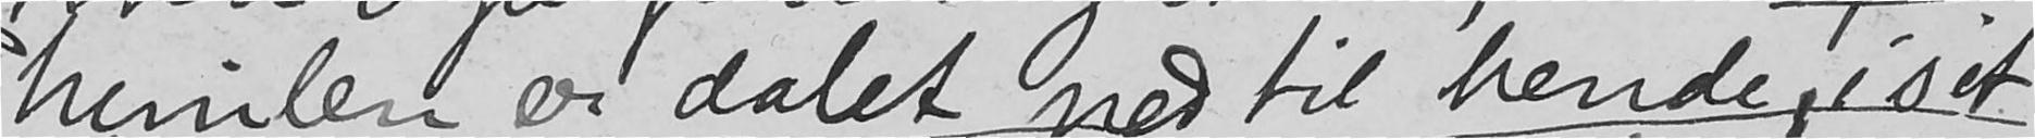himlen er dalet ned til hende, i sit
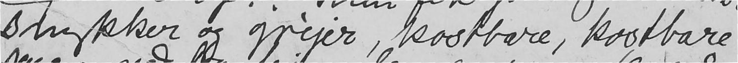smykker og grejer, kostbare, kostbare
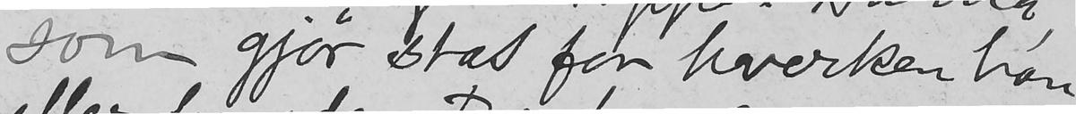som gjør stas for hverken han
